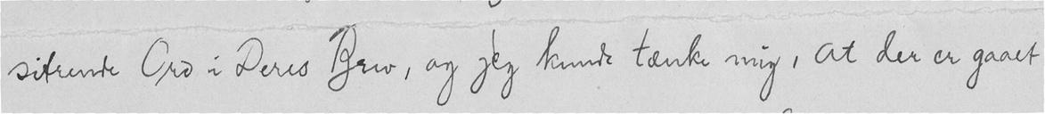sitrende Ord i Deres Brev, og jeg kunde tænke mig, at der er gaaet
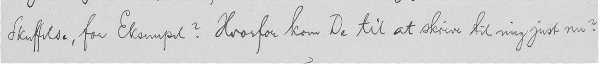Skuffelse, for Eksempel? Hvorfor kom De til at skrive til mig just nu?
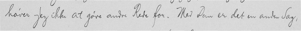høver jeg ikke at gøre andre Rede for. Med Dem er det en anden Sag,
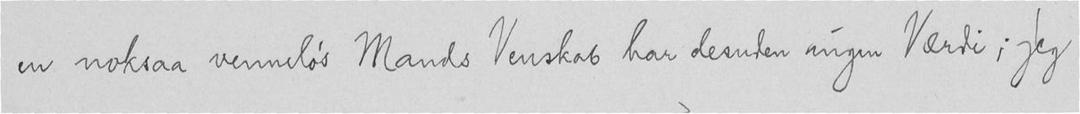en noksaa venneløs Mands Venskab har desuden ingen Værdi; jeg
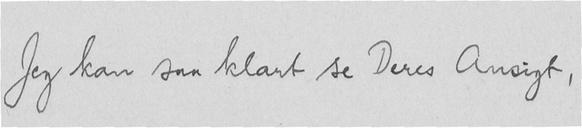Jeg kan saa klart se Deres Ansigt,
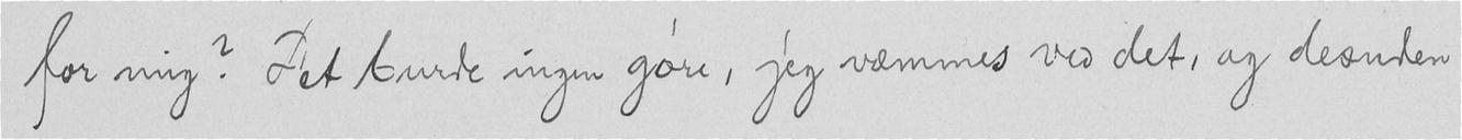for mig? Det burde ingen gøre, jeg væmmes ved det, og desuden
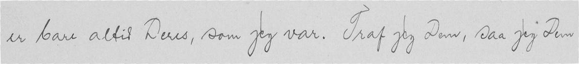er bare altid Deres, som jeg var. Traf jeg Dem, saa jeg Dem
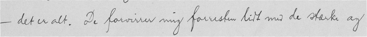- det er alt. De forvirrer mig forresten lidt med de stærke og
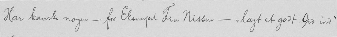Har kanske nogen - for Eksempel Fru Nissen - "lagt et godt Ord ind"
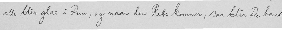alle blir glad i Dem, og naar den Rette kommer, saa blir De hans
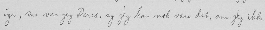igen, saa var jeg Deres, og jeg kan nok være det, om jeg ikke
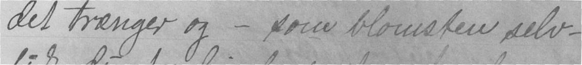det trænger og - som blomsten selv -
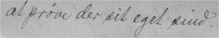at prøve der sit eget sind.
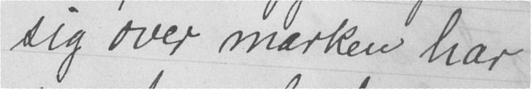sig over marken har
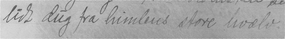lidt dug fra himlens store hvælv.
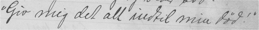"Giv mig det alt indtil min død!"
